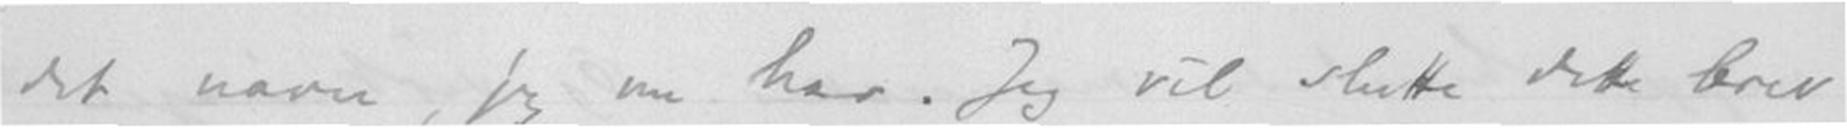det navn, jeg nu har. Jeg vil slutte dette brev
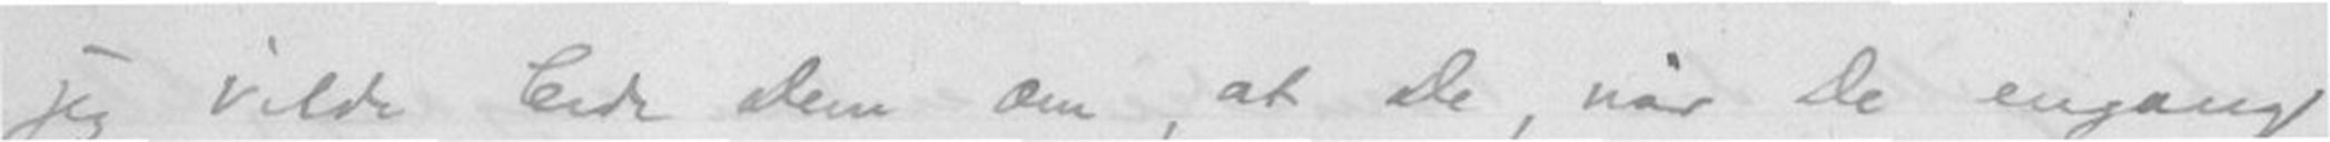jeg vilde bede Dem om, at De, når De engang
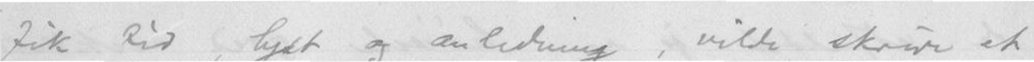fik tid, lyst og anledning, vilde skrive et
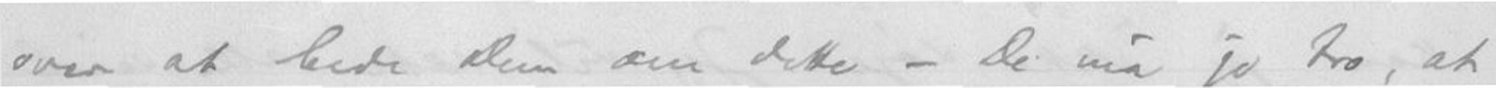over at bede Dem om dette - De må jo tro, at
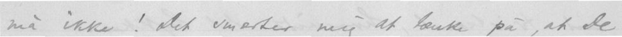må ikke! Det smerter mig at tænke på, at De
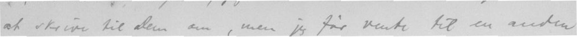at skrive til Dem om, men jeg får vente til en anden
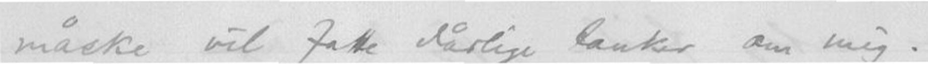måske vil fatte dårlige tanker om mig.
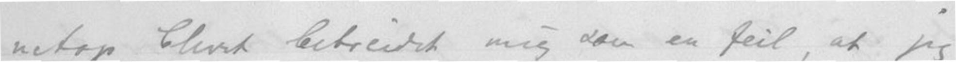netop blivet bebreidet mig som en feil, at jeg
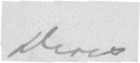Deres
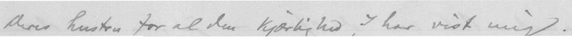Deres hustru for den kjærlighed, I har vist mig.
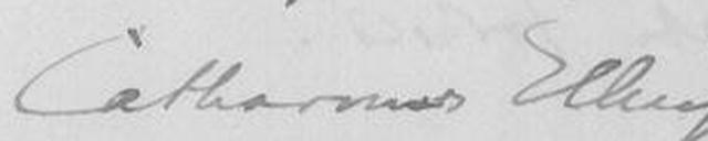Catharinus Elling
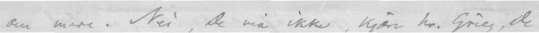om mere. Nei, De må ikke, kjære hr. Grieg, De
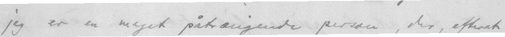jeg er en meget påtrængende persom. der, efterat
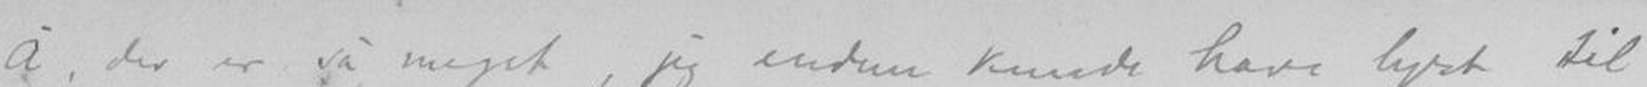Å, der er så meget, jeg endnu kunde have lyst til
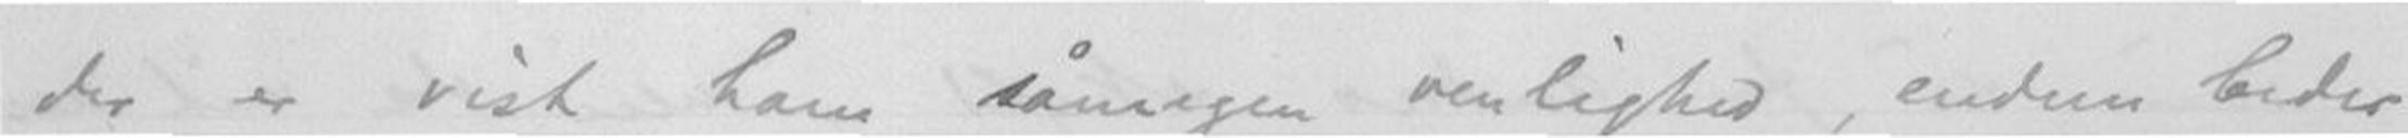der er vist ham såmegen venlighed, endnu beder
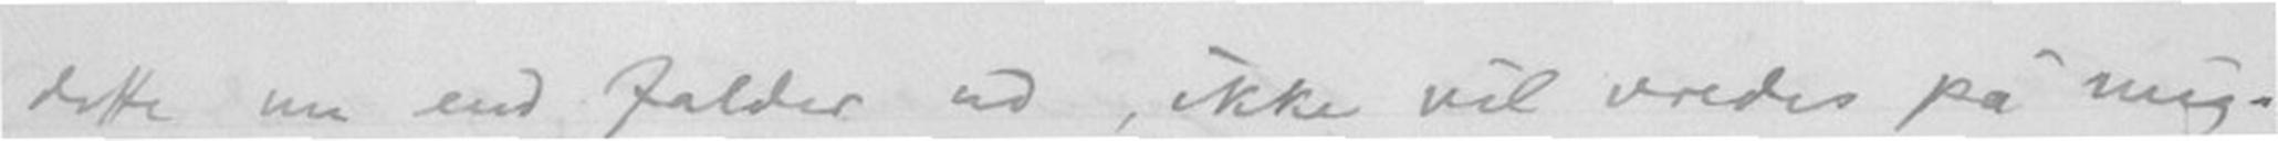dette nu end falder ud, ikke vil vredes på mig.
